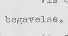begavelse.
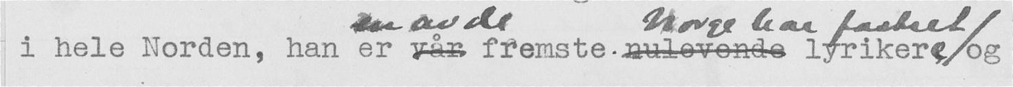i hele Norden, han er vår en av de fremste nulevende lyrikere Norge har forstret
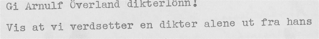Vis at vi verdsetter en dikter alene ut fra hans
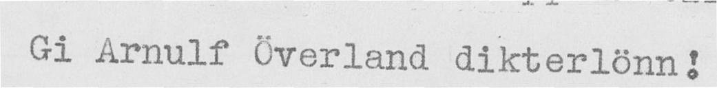Gi Arnulf Överland dikterlönn!
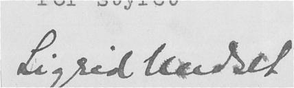Sigrid Undset
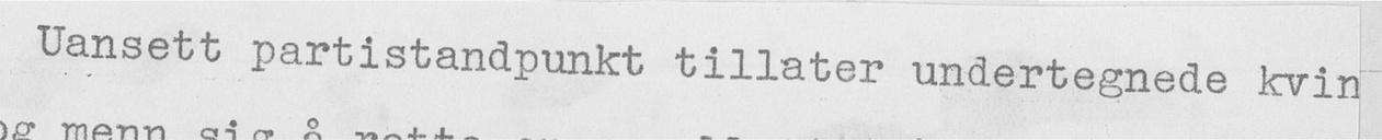Uansett partistandpunkt tillater undertegnede kvin
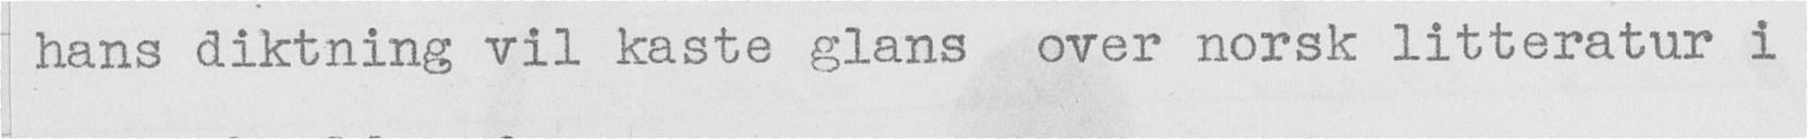hans diktning vil kaste glans over norsk litteratur i
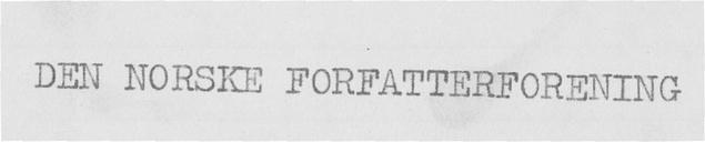DEN NORSKE FORFATTERFORENING
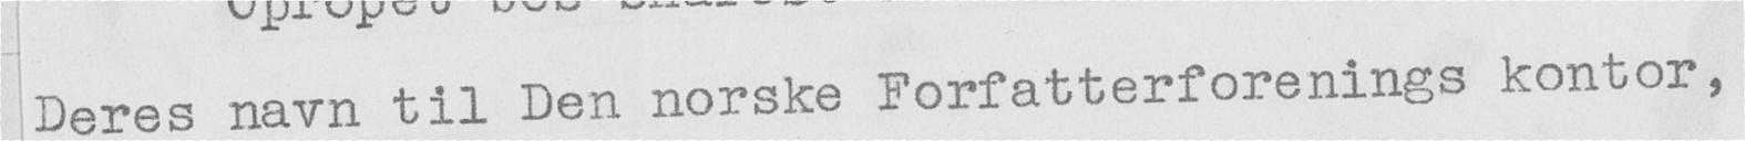Deres navn til Den norske Forfatterforenings kontor,
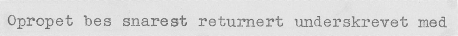Opropet bes snarest returnert underskrevet med
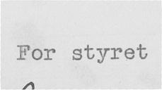For styret
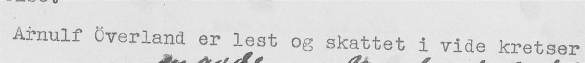Arnulf Överland er lest og skattet i vide kretser
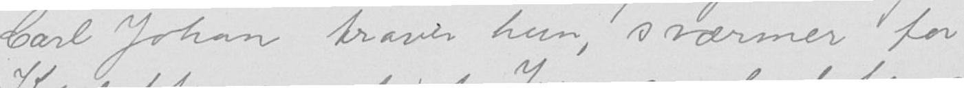Carl Johan traver hun, sværmer for
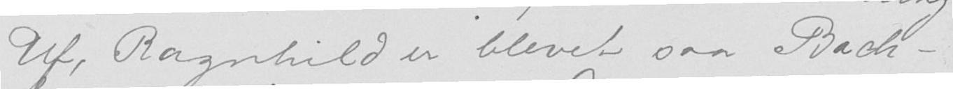Uf, Ragnhild er blevet saa Back-
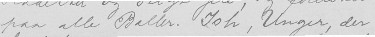paa alle Baller. Ish, Unger, der
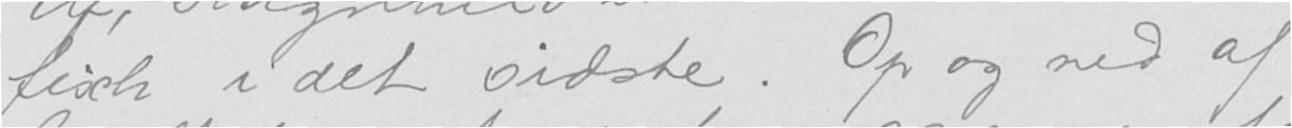fisch i det sidste. Op og ned af
